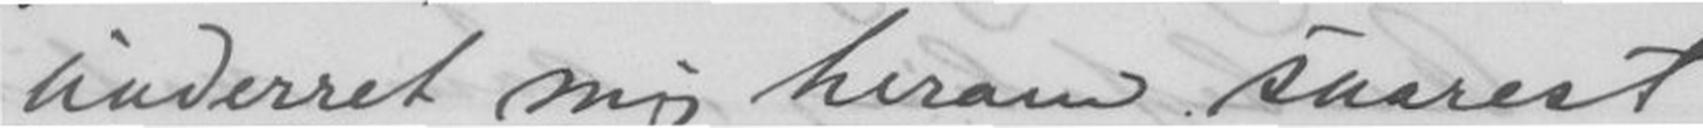underret mig herom snarest
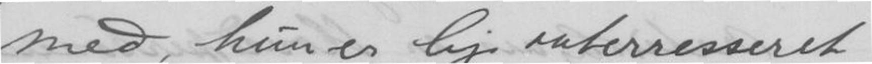med, hun er lige interresseret
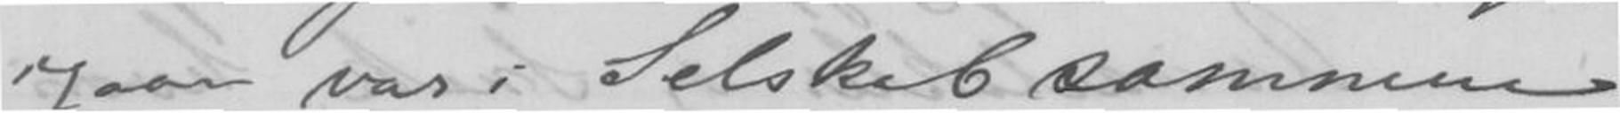ogsaa var i Selskab sammen
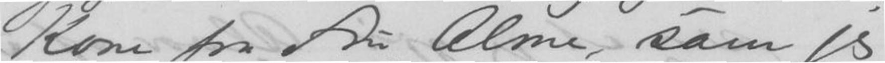Kone fra Fru Alme, som jeg
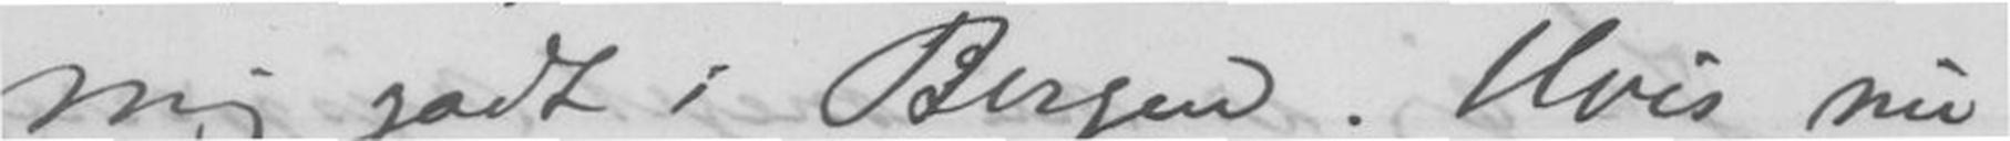mig godt i Bergen. Hvis nu
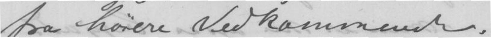fra høiere Vedkommende.
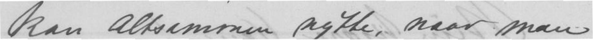kan Altsammen nytte, naar man
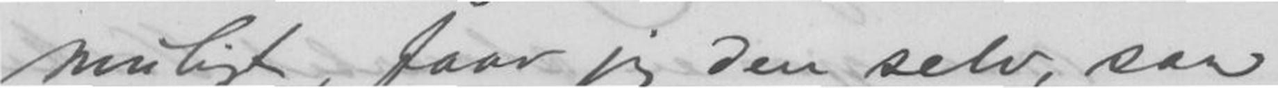muligt, faar jeg den selv, saa
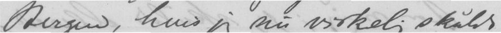Bergen, hvis jeg nu virkelig skald
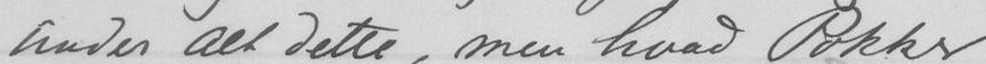Under Alt dette, men hvad Pokker
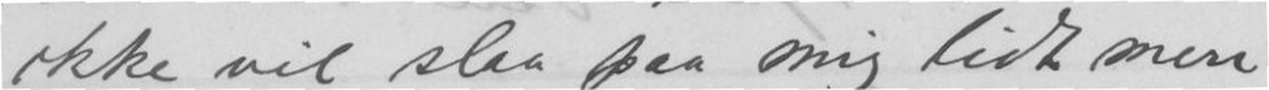ikke vil slaa paa mig lidt mere
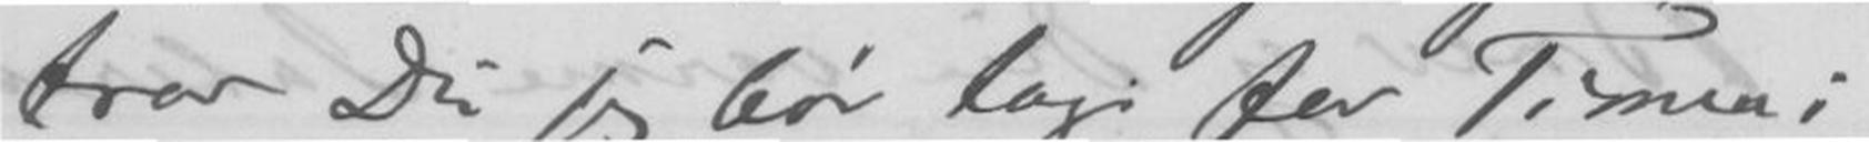tror Du jeg bør tage for Timen i
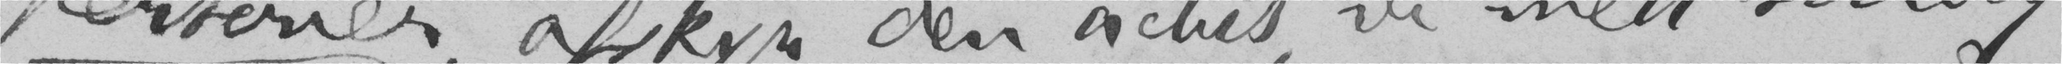personer afskyr den actus vi med smag
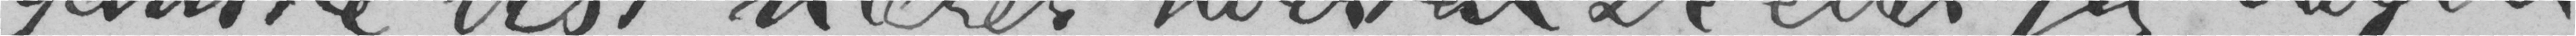Ganske vist nærer hverken De eller jeg nogen
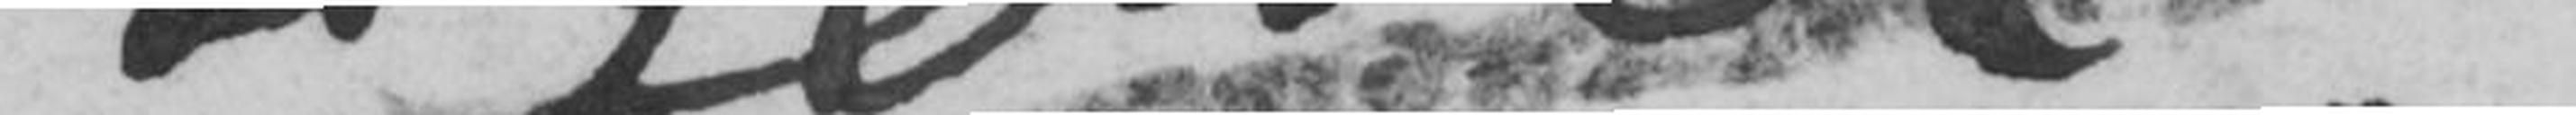sigelser.
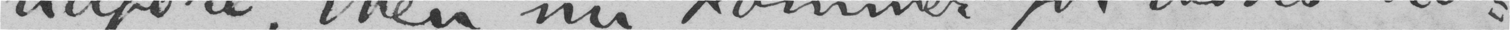udføre. Men nu kommer for disses ved-
